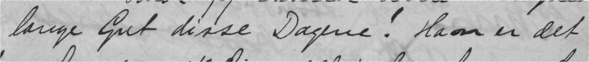lange Gut disse dagene! Han er det
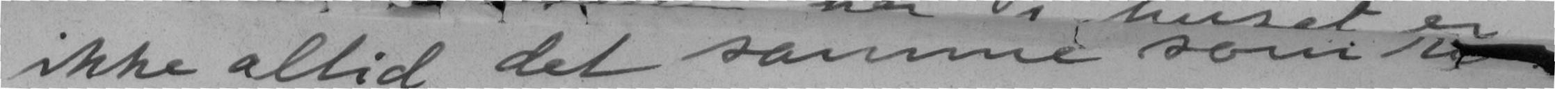ikke altid det samme som ro
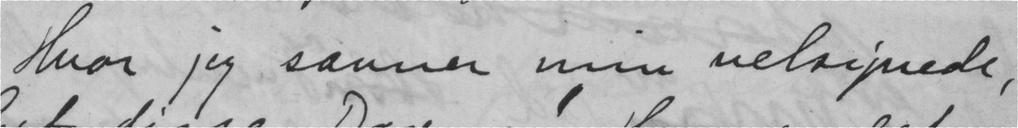Hvor jeg savner min velsignede,
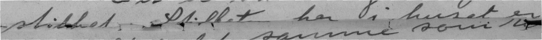stilhet. Stilhet her i huset er
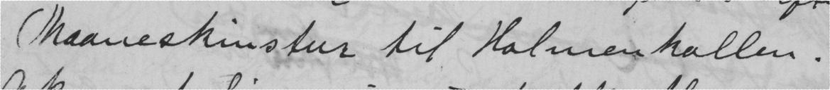Maaneskinstur til Holmenkollen.
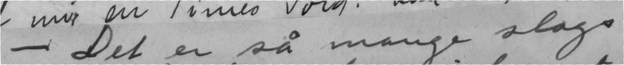- Det er så mange slags
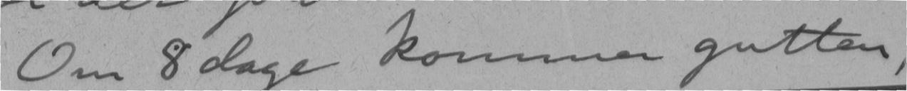Om 8 dage kommer gutten,
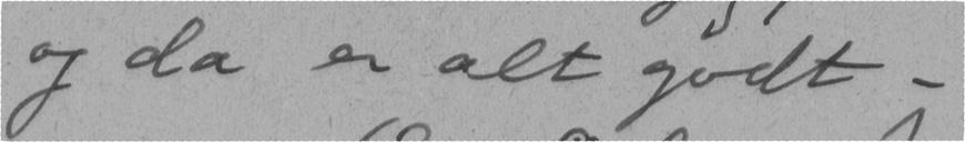og da er alt godt -
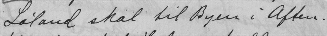Løland skal til Byen i aften.
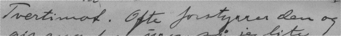Tvertimot. Ofte forstyrrer den og
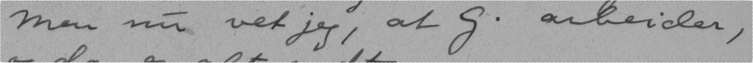men nu vet jeg, at G. arbeider,
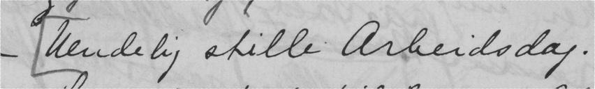- Uendelig stille arbeidsdag.
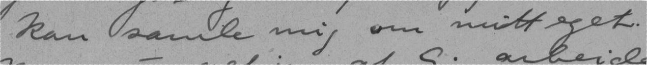kan samle mig om mitt eget.
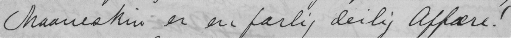Maaneskin er en farlig deilig affære!
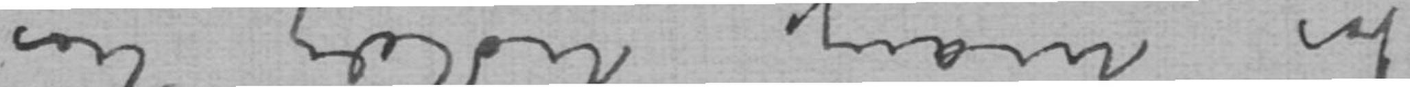for mange udlæg har
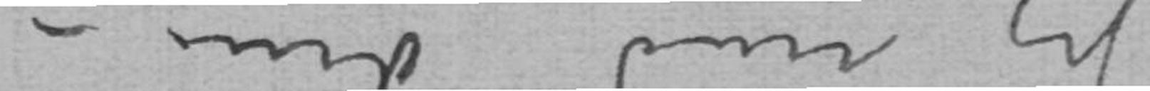jeg med dem –
